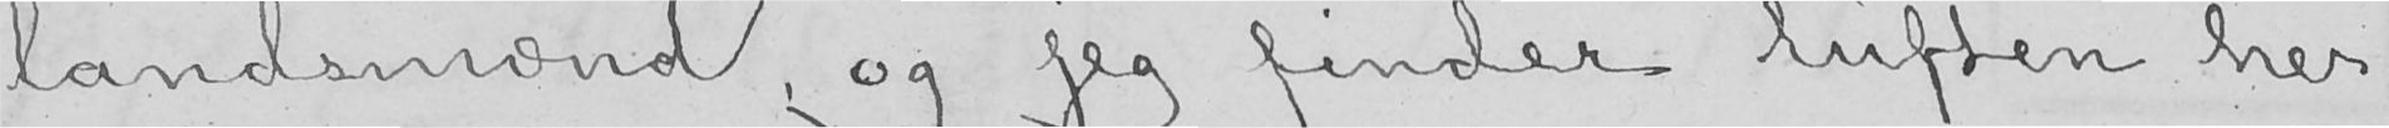landsmænd, og jeg finder luften her-
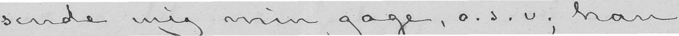sende mig min gage, o. s. v; han
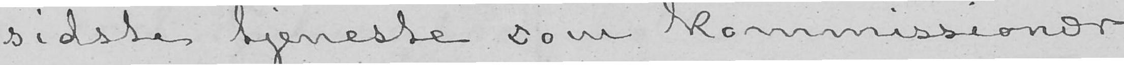sidste tjeneste som kommissionær
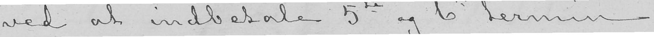ved at indbetale 5te og 6te termin
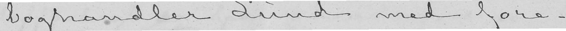boghandler Lund med fore-
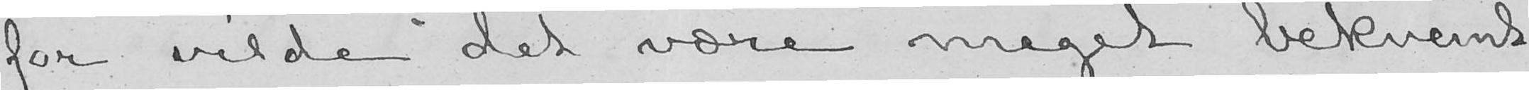for vilde det være meget bekvemt
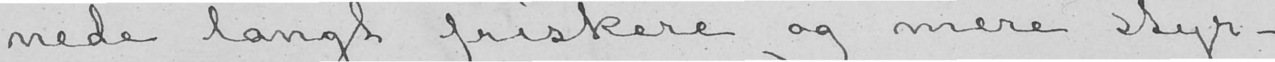nede langt friskere og mere styr-
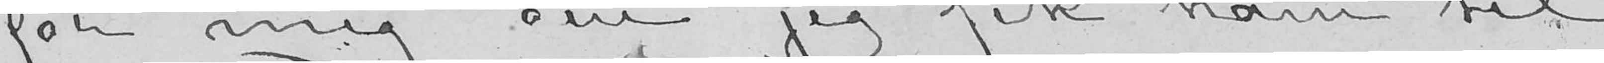for mig om jeg fik ham til
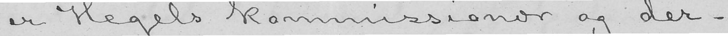er Hegels kommissionær og der-
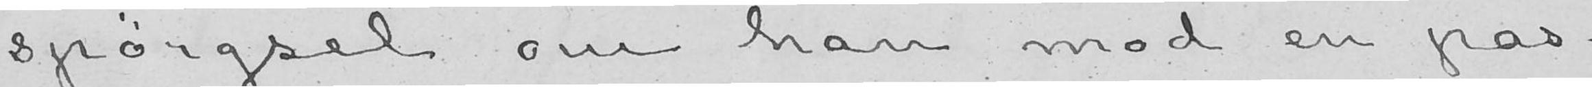spørgsel om han mod en pas-
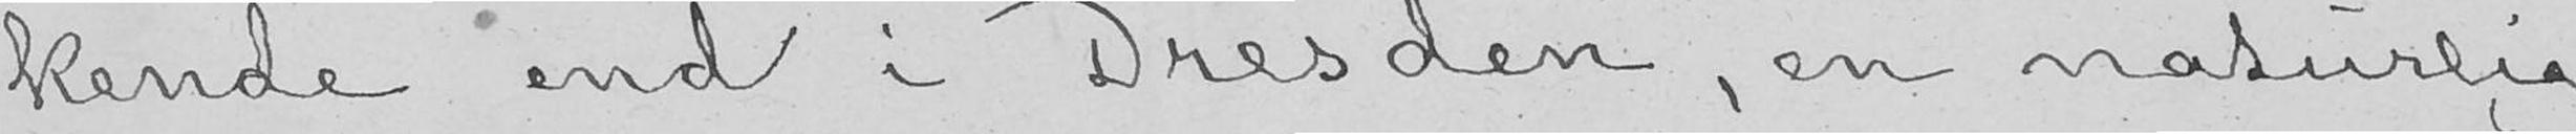kende end i Dresden, en naturlig
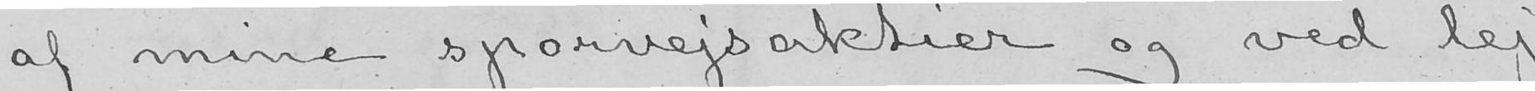af mine sporvejsaktier og ved lej-
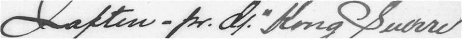Iaften - pr. d/. "Kong Sverre
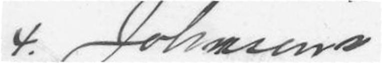4. Johnsens
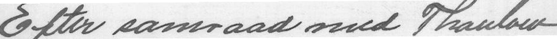Efter samraad med Thaulow
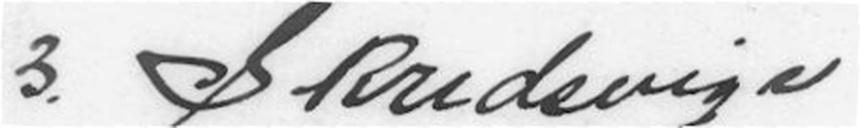3. Skredsvigs
